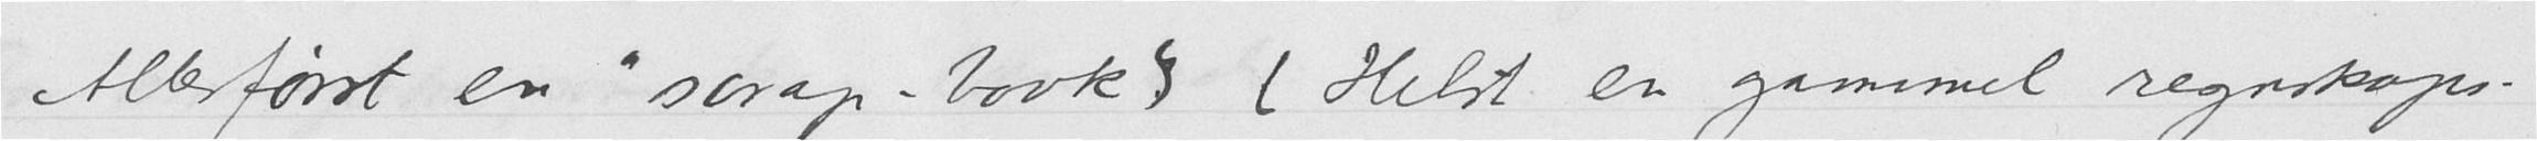Allerførst en "scrap-book" (Helst en gammel regnskaps-
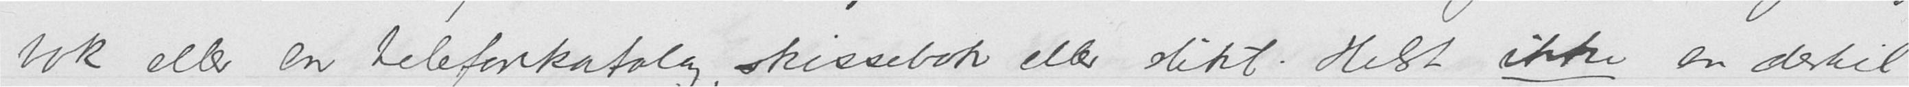bok eller en telefonkatalog, skissebok eller slikt. Helst ikke en dertil
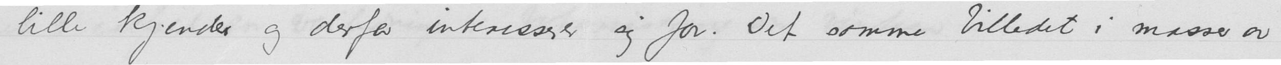lille kjender og derfor interesserer sig for. Det samme billedet i masser av
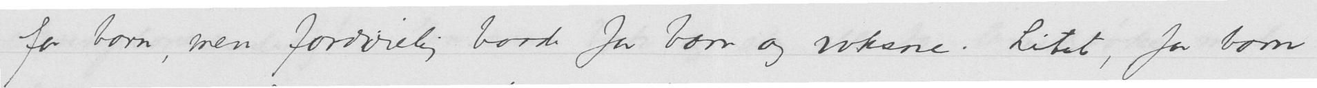for barn, men fordøielig baade for barn og voksne. Litet, for barn
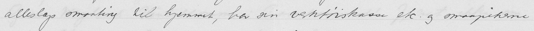alleslags smaating til hjemmet, har sin verktøiskasse etc. og smaapikerne
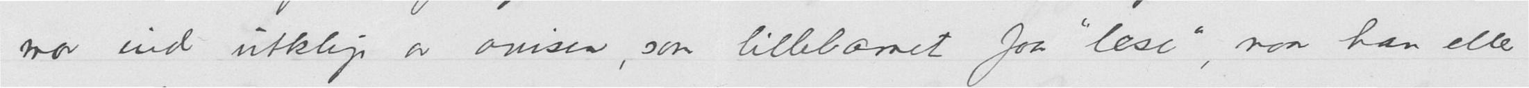mor ind utklip av avisen, som lillebarnet faar "lese", naar han eller
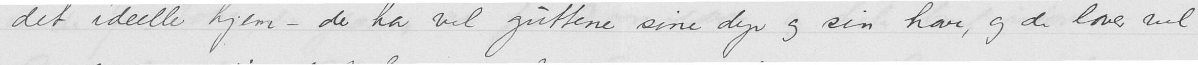det ideelle hjem - der har vel guttene sine dyr og sin have, og de laver vel
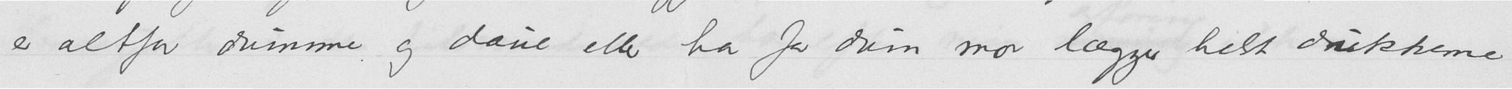er altfor dumme og daue eller har for dum mor lægger helst dukkerne
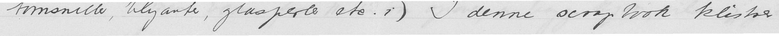tomsneller, blyanter, glasperler etc.i) I denne scrapbook klistrer
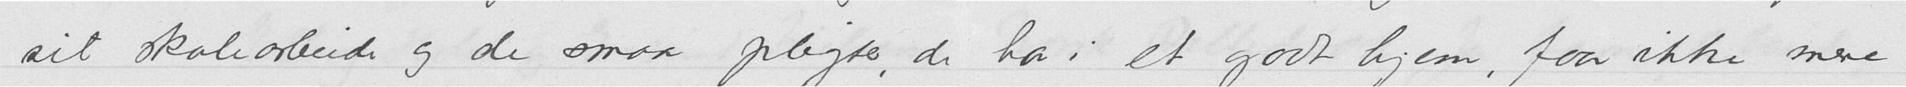sit skolearbeide og de smaa pligter, de har i et godt hjem, faar ikke mere
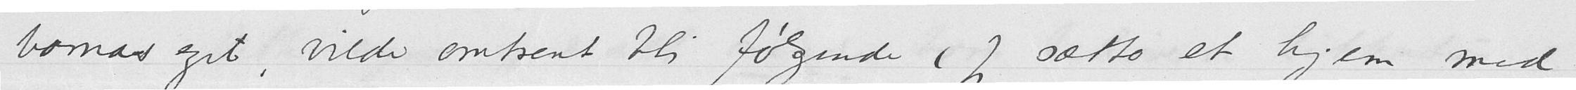barnas eget, vilde omtrent bli følgende (Jeg sætter et hjem med
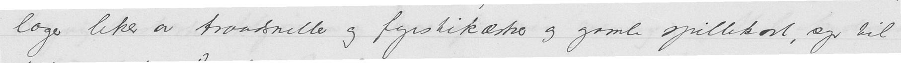lager leker av traadsneller og fyrstikæsker og gamle spillekort, syr til
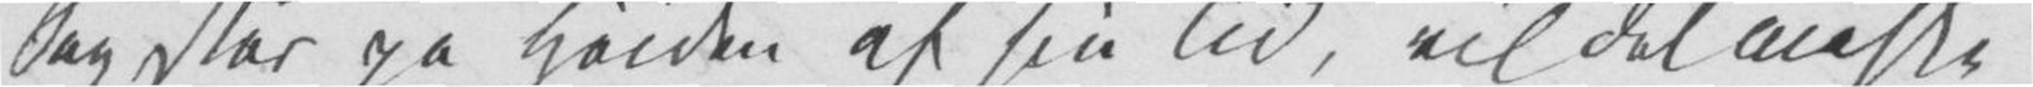Sag står på Høiden af sin Tid, vil det måske
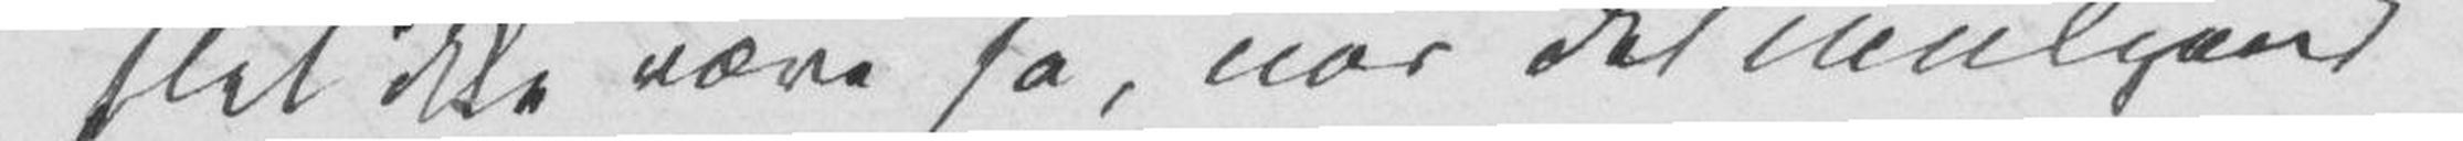slet ikke være så, når det muligvis
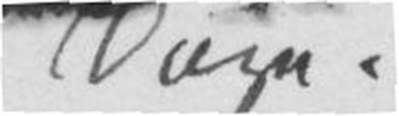Døgn.
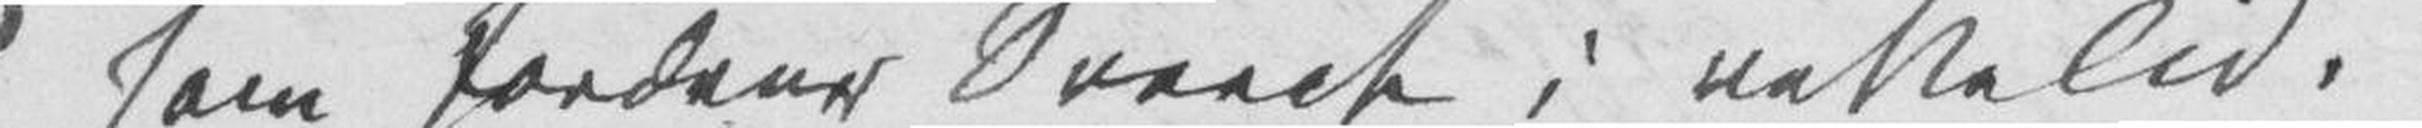som fordrer Sverdet i rette Tid.
